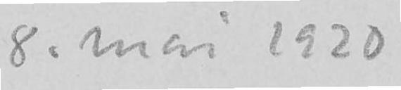8.mai.1920
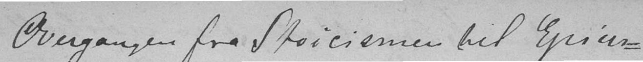Overgangen fra Stoicismen til Epicu-
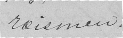ræismen.
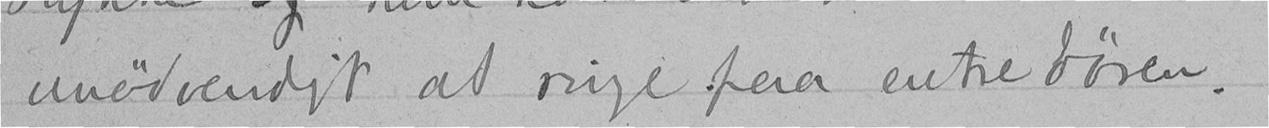unødvendigt at ringe paa entredøren.
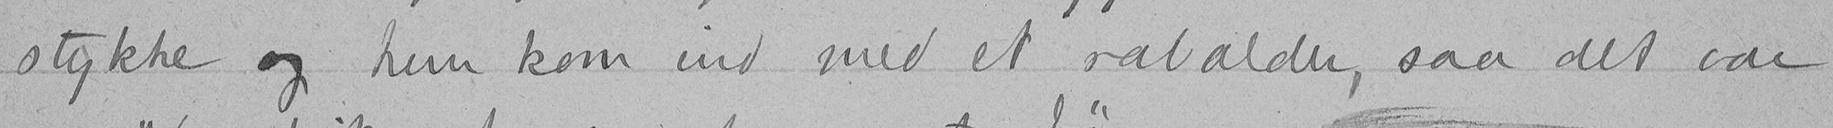stykke og hun kom ind med et rabalder, saa det var
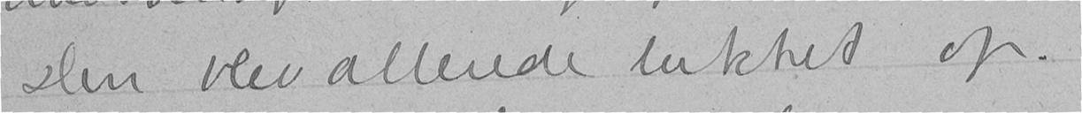Den blev allerede lukket op.
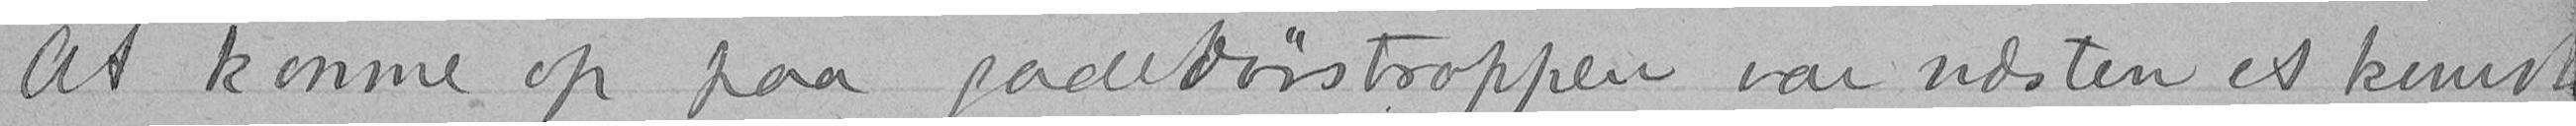At komme op paa gadedørstrappen var næsten et kunst
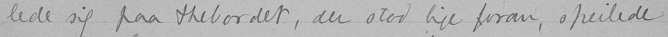lede sig paa thebordet, der stod lige foran, speilede
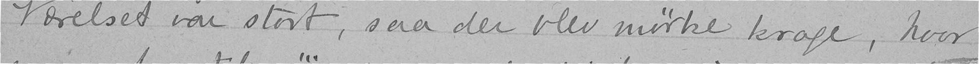Værelset var stort, saa der blev mørke kroge, hvor
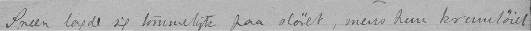Sneen lagde sig tommetyk paa sløret, mens hun krumbøiet
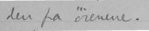den fra øienene.
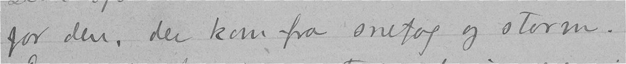for den, der kom fra snefog og storm.
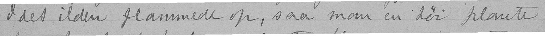Idet ilden flammede op, saa man en høi plante
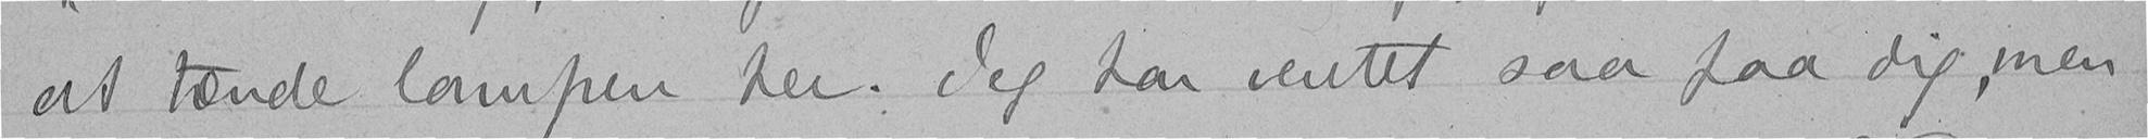at tænde lampen her. Jeg har ventet saa paa dig, men
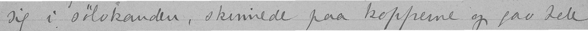sig i sølvkanden, skinnede paa kopperne og gav hele
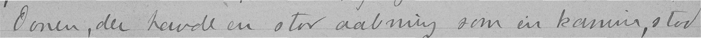Oonen, der havde en stor aabning som en kamin, stod
